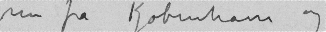nu fra Kjøbenhavn og
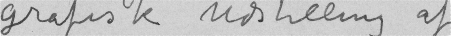Grafisk Udstilling af
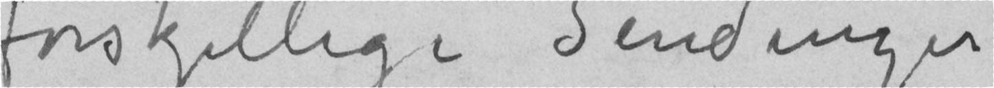forskjellige Sendinger
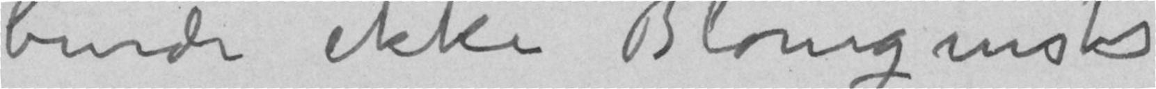burde ikke Blomqvists
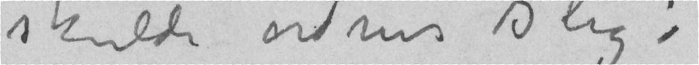skulde ordnes slig:
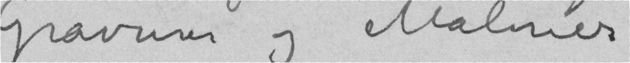Gravurer og Malerier
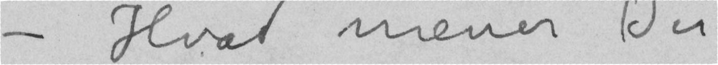– Hvad mener Du
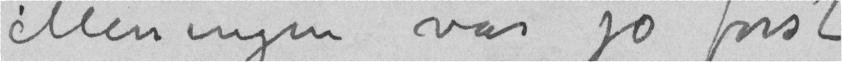Meningen var jo først
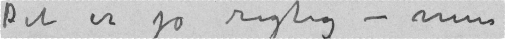Det er jo rigtig – Men
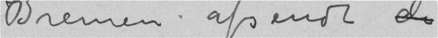Bremen afsendt de
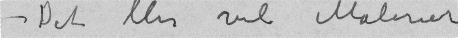– Det blir vel Malerier
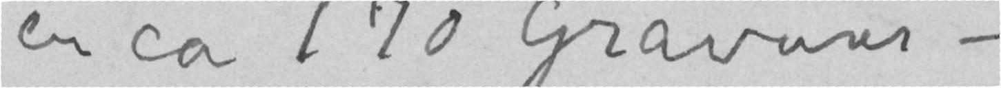en ca 170 Gravurer –
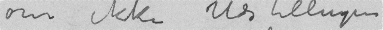om ikke Udstillingen
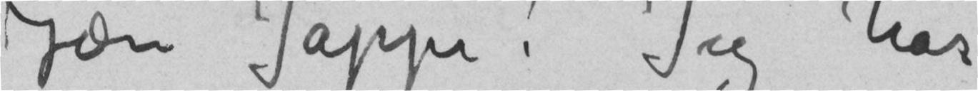Kjære Jappe! Jeg har
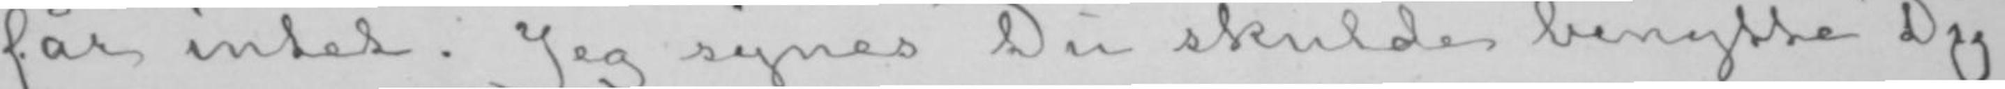får intet. Jeg synes Du skulde benytte Dig
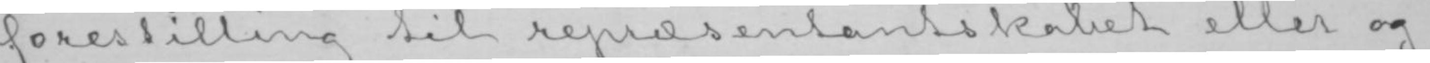forestilling til repræsentantskabet eller og-
